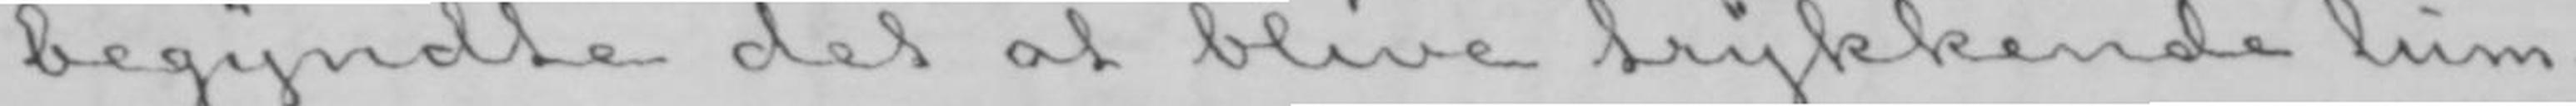begyndte det at blive trykkende lum-
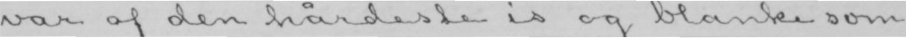var af den hårdeste is og blanke som
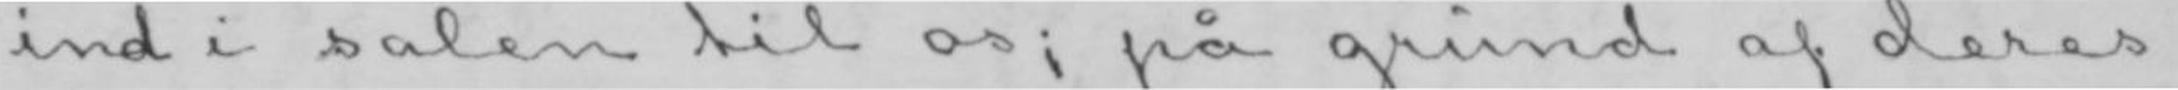ind i salen til os; på grund af deres
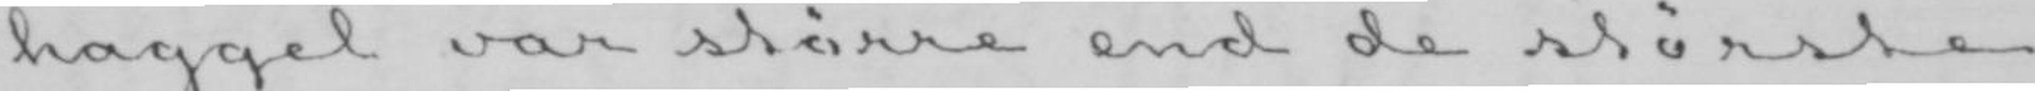haggel var større end de største
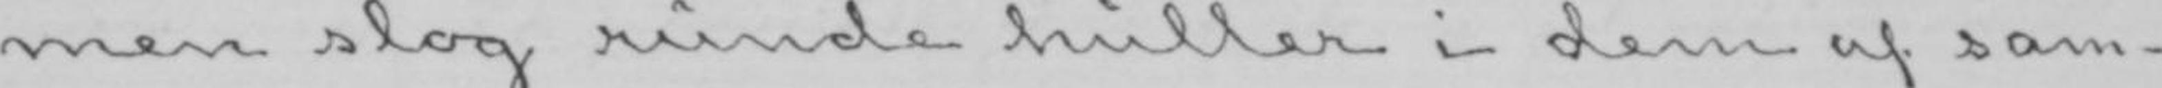men slog runde huller i dem af sam-
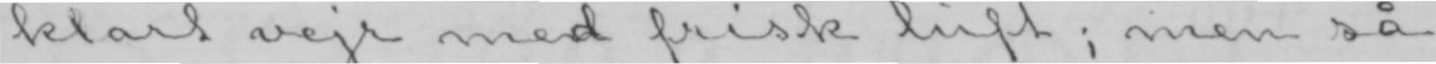klart vejr med frisk luft; men så
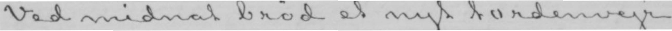Ved midnat brød et nyt tordenvejr
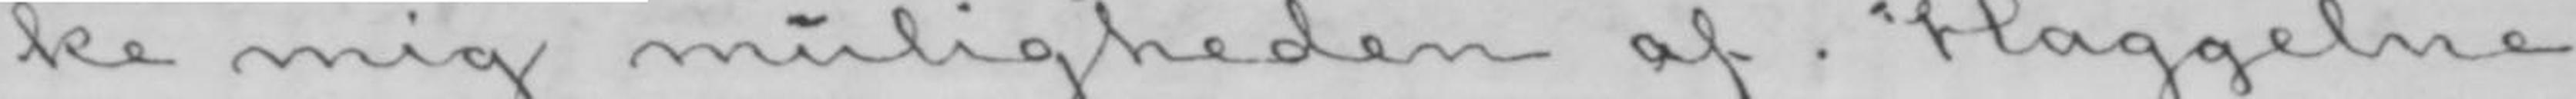ke mig muligheden af. Haggelne
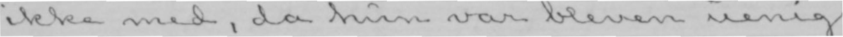ikke med, da hun var bleven uenig
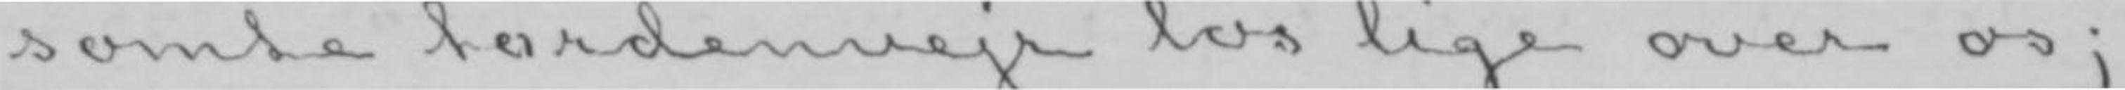somte tordenvejr løs lige over os;
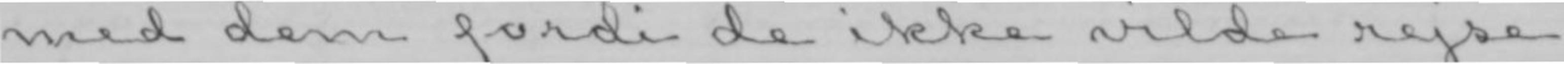med dem fordi de ikke vilde rejse
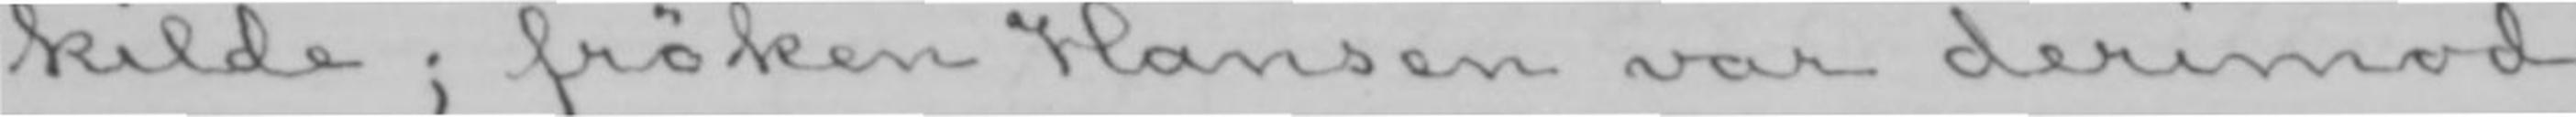kilde; frøken Hansen var derimod
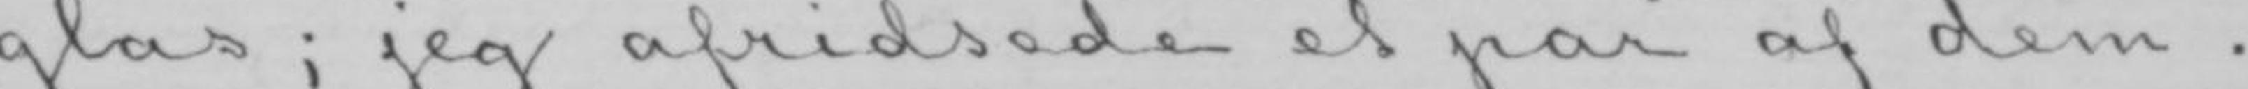glas; jeg afridsede et par af dem.
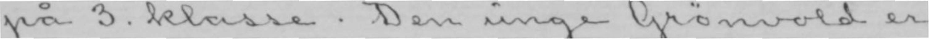på 3. klasse. Den unge Grønvold er
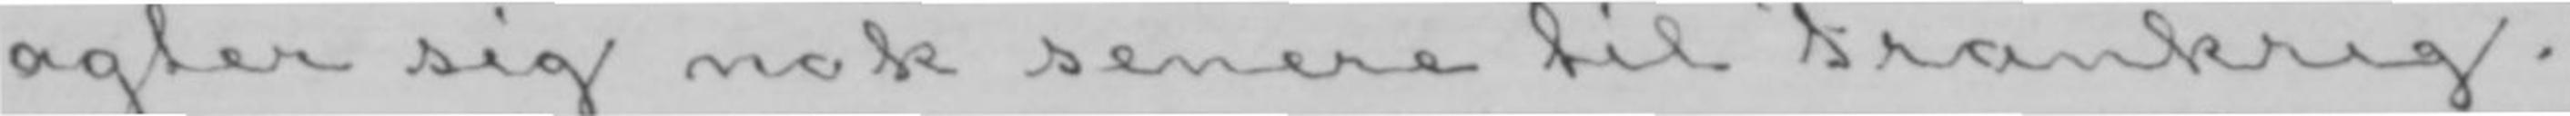agter sig nok senere til Frankrig.
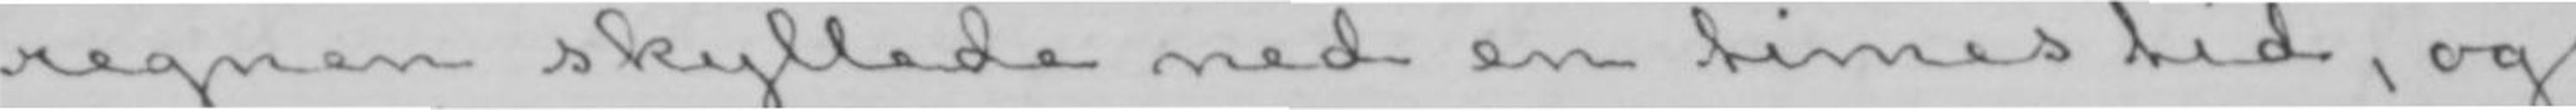regnen skyllede ned en times tid, og
